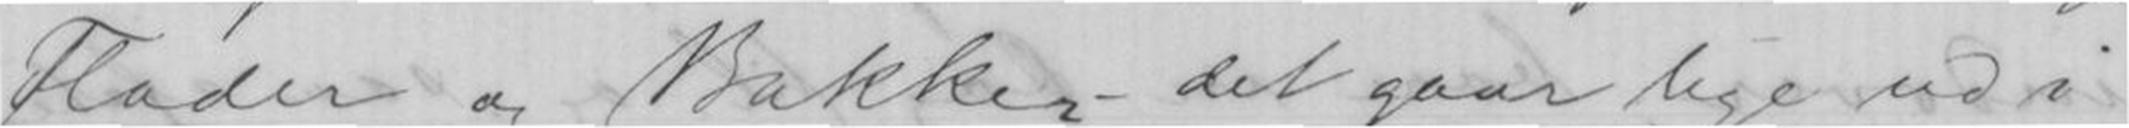Flader og Bakker - det gaar lige ud i
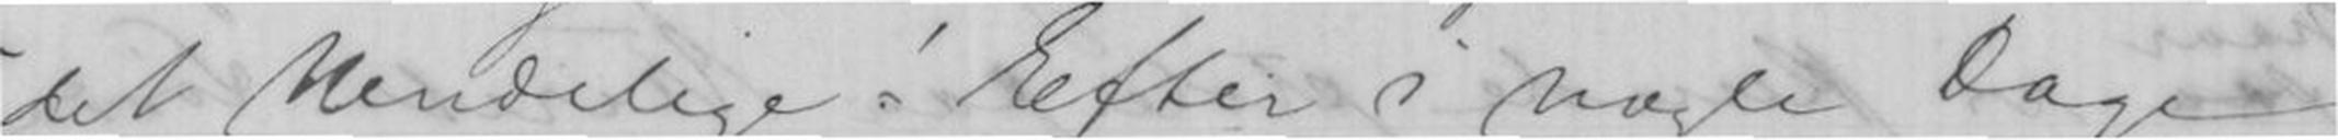det Uendelige! Efter i nogle Dage
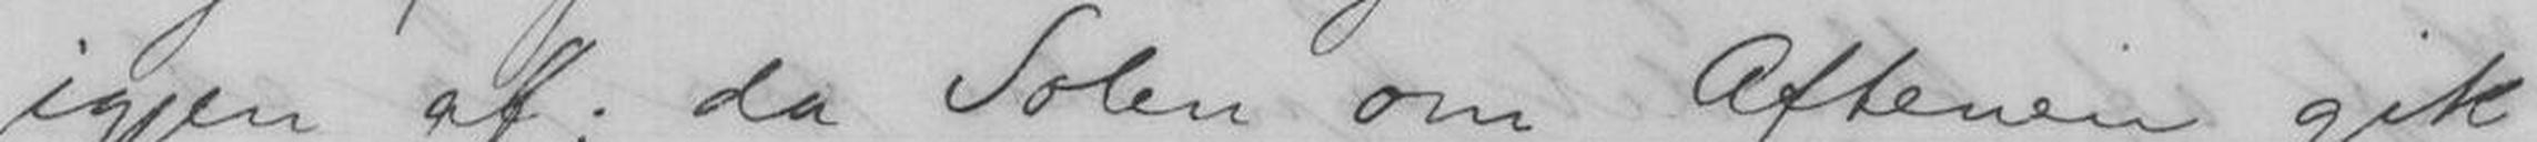igjen af; da Solen om Aftenen gik
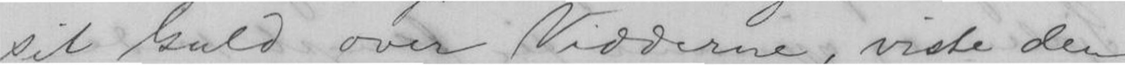sit Guld over Vidderne, viste den
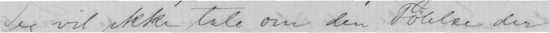Jeg vil ikke tale om den Følelse der
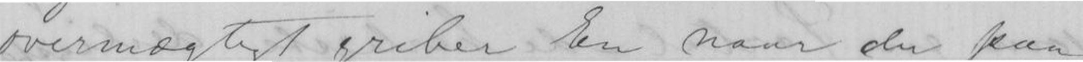overmægtigt griber En naar du paa
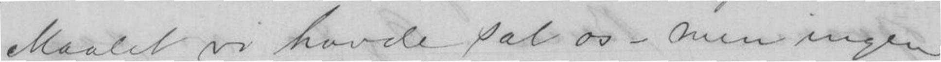Maalet vi havde sat os - men ingen
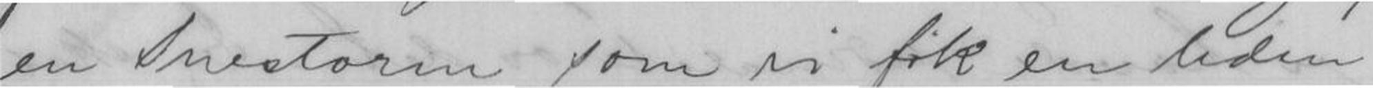en Snestorm, som vi fik en liden
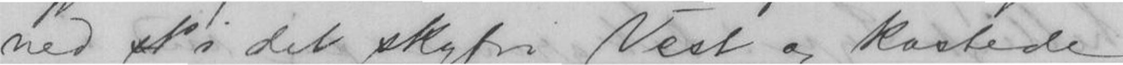ned sk i det skyfri Vest og kastede
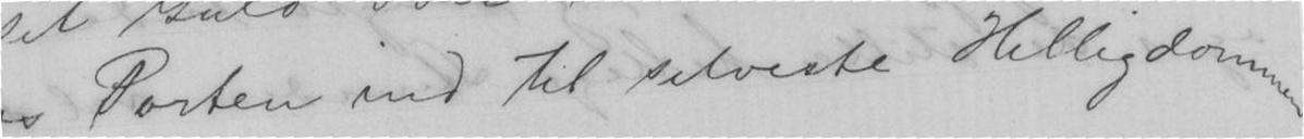os Porten ind til selveste Helligdommen
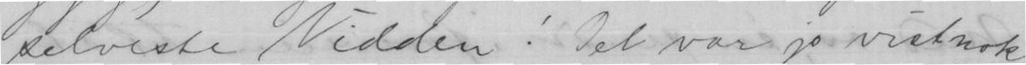selveste Vidden! Det var jo vistnok
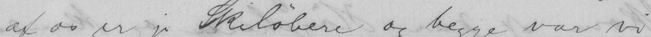af os er jo Skiløbere og begge var vi
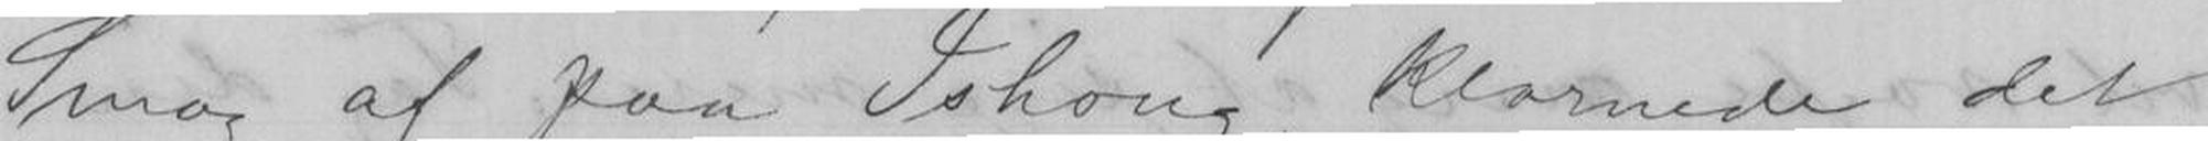Smag af paa Ishoug, klarnede det
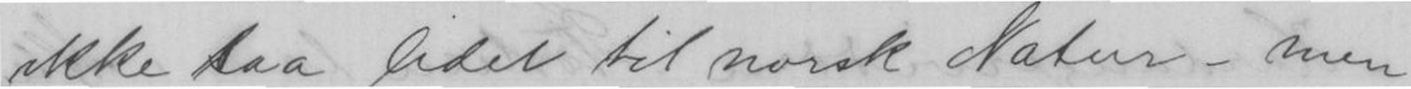ikke saa lidet til norsk Natur - men
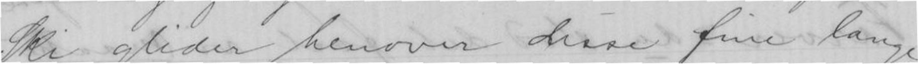Ski glider henover disse fine lange
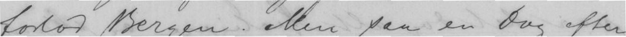forlod Bergen. Men saa en Dag efter
